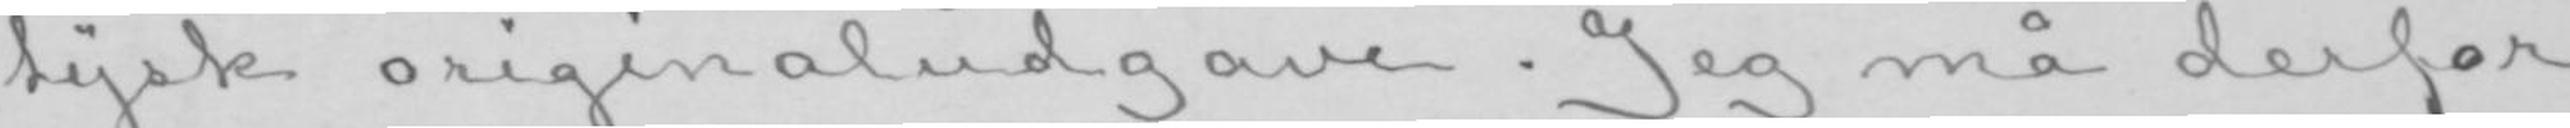tysk originaludgave. Jeg må derfor
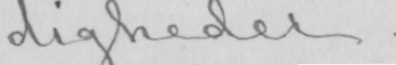digheder.
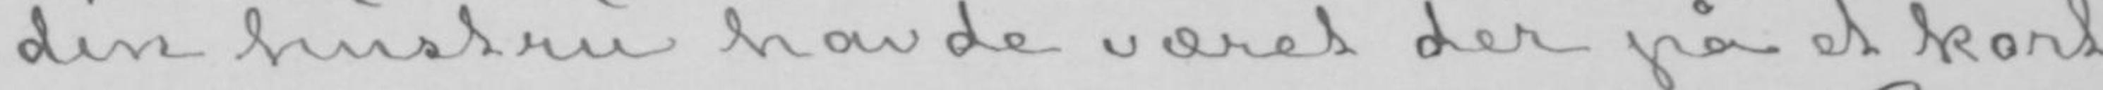din hustru havde været der på et kort
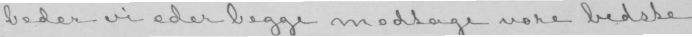beder vi eder begge modtage vore bedste
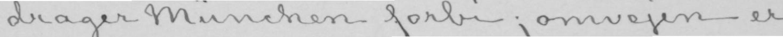drager München forbi; omvejen er
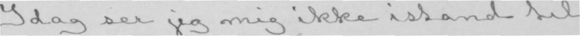Idag ser jeg mig ikke istand til
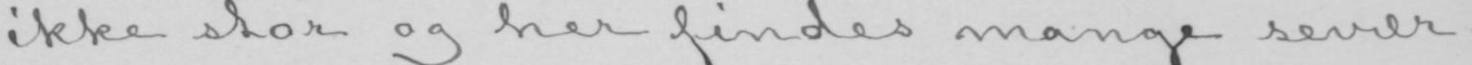ikke stor og her findes mange sevær-
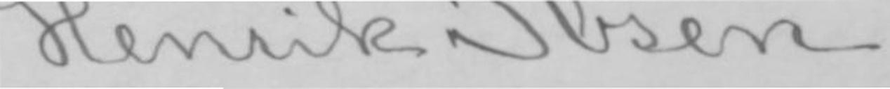Henrik Ibsen.
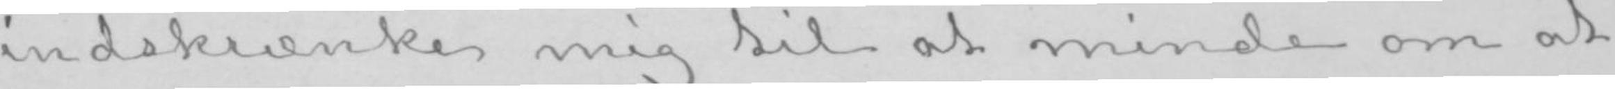indskrænke mig til at minde om at
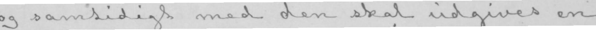og samtidigt med den skal udgives en
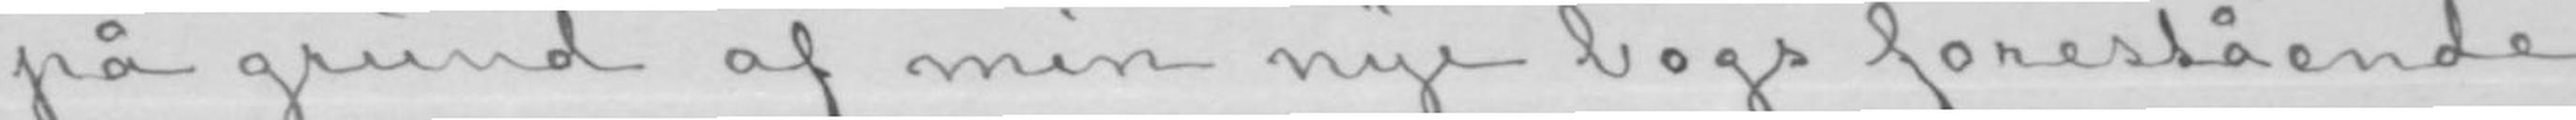på grund af min nye bogs forestående
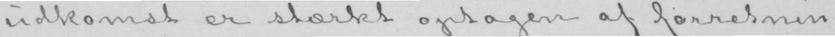udkomst er stærkt optagen af forretnin-
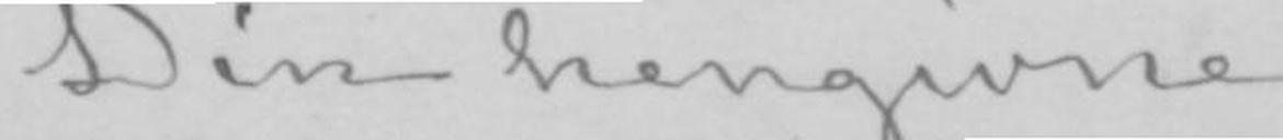Din hengivne
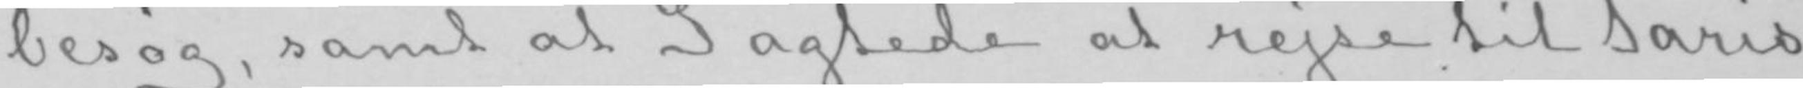besøg, samt at I agtede at rejse til Paris
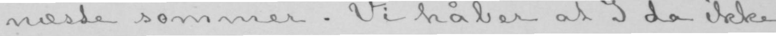næste sommer. Vi håber at I da ikke
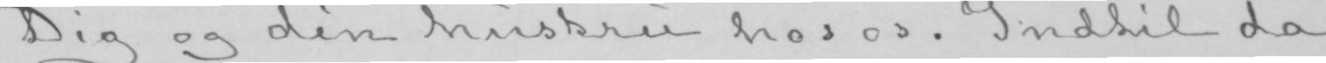Dig og din hustru hos os. Indtil da
